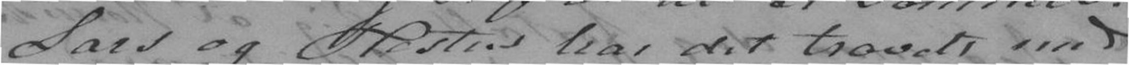Lars og Hesten har det travelt med
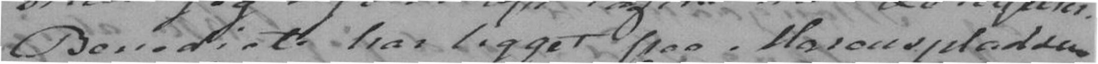Benedicte har ligget paa Marenspladsen
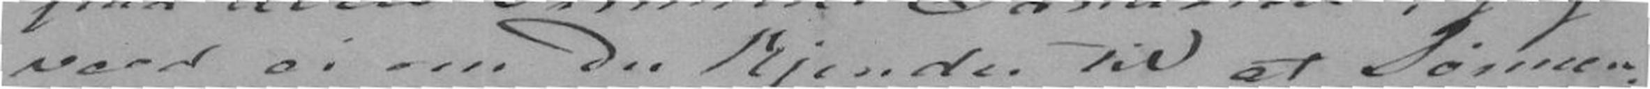veed ei nu Du kjender til at Sønnen
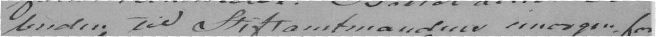buden til Stiftamtmandens imorgen for
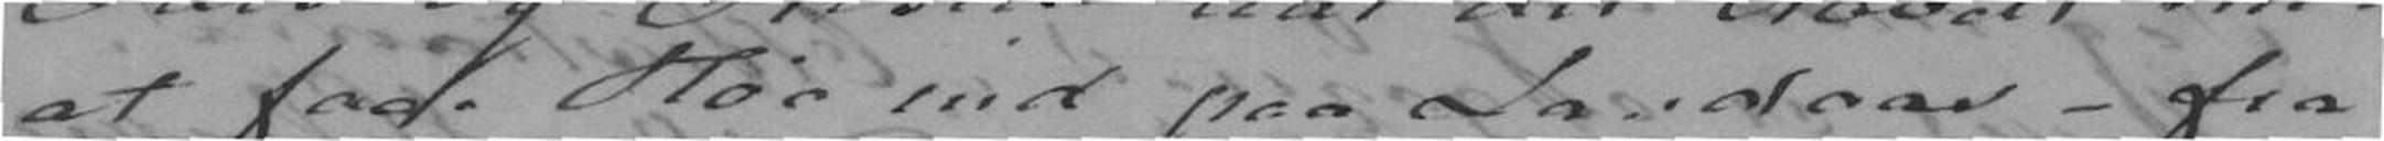at faae Høe ind paa Landaas - fra
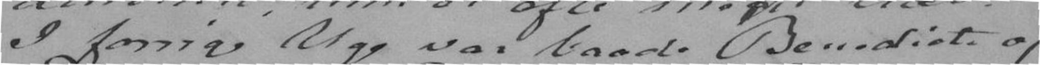I forrige Uge var baade Benedicte og
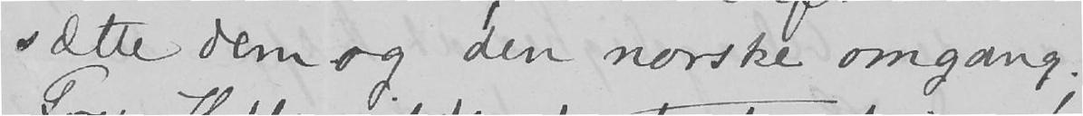sætte dem og den norske omgang.
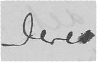Deres
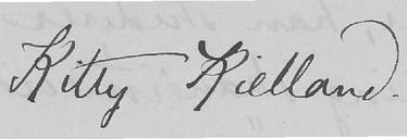Kitty Kielland.
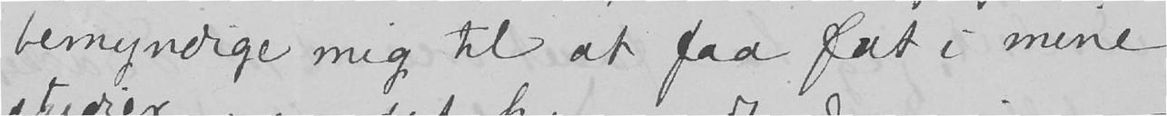bemyndige mig til at faa fat i mine
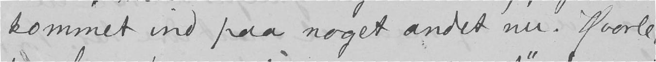kommet ind paa noget andet nu. Hvorle
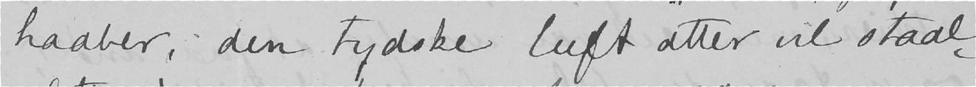haaber, den tydske luft atter vil staal
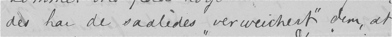des har de saaledes «verweichert» dem, at
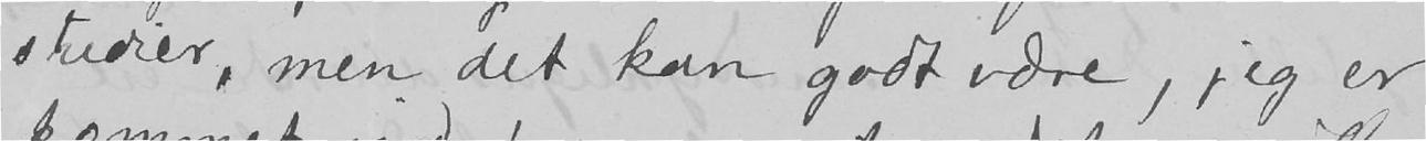studier, men det kan godt være, jeg er
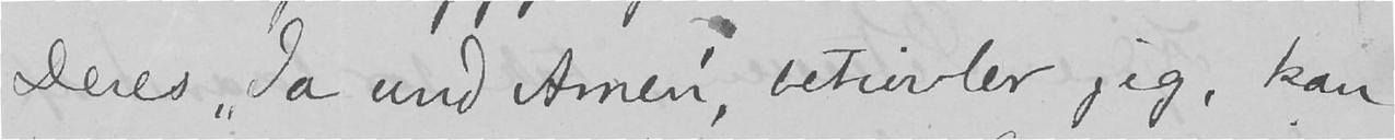Deres «Ja und Amen», betvivler jeg, kan
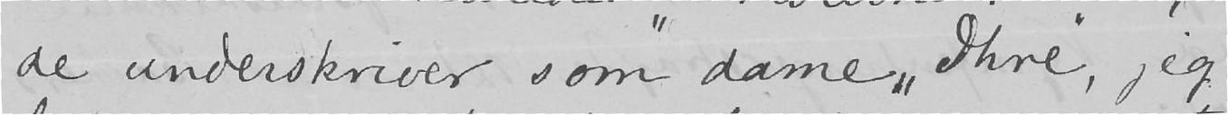de underskriver som dame «Ihre», jeg
